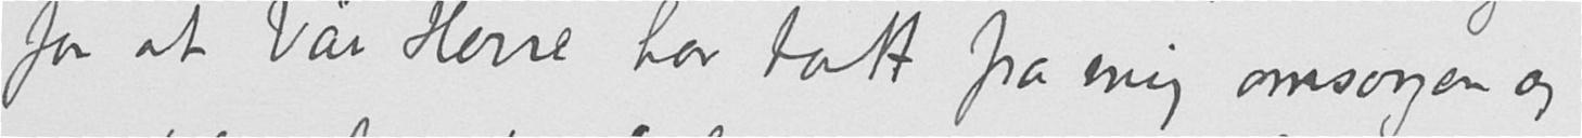for at Vår Herre har tatt fra mig omsorgen og
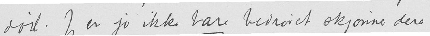død. Jeg er jo ikke bare bedrøvet skjønner dere
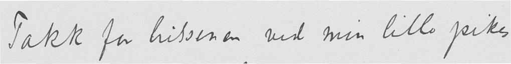Takk for hilsenen ved min lille pikes
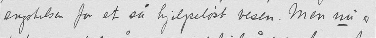engstelsen for et så hjelpeløst vesen. Men nu er
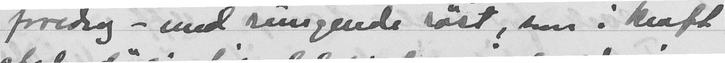foredrag - med rungende røst, som i kraft
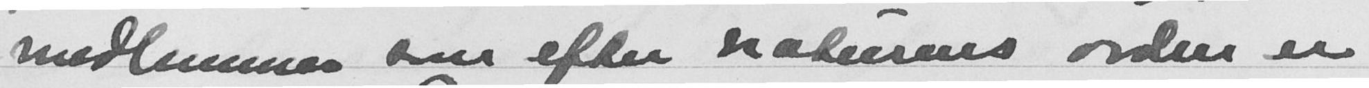medlemmer som efter naturens orden er
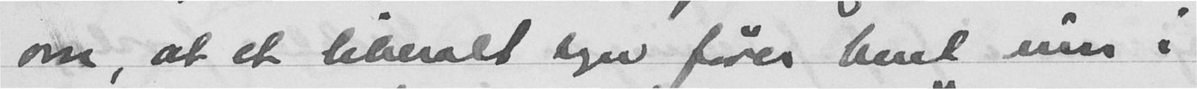om, at et liberalt syn fører bent inn i
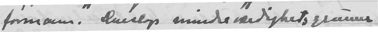formann. Denslags mindreverdighetsgrunner
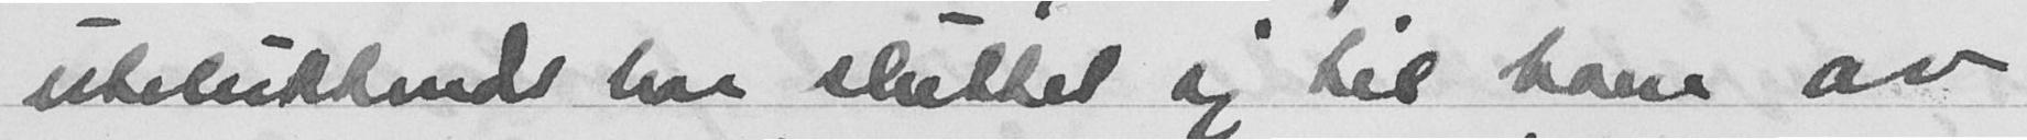utelukkende har sluttet sig til ham av
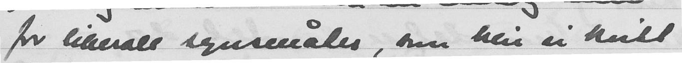for liberale synsmåter, som blir vi kvitt
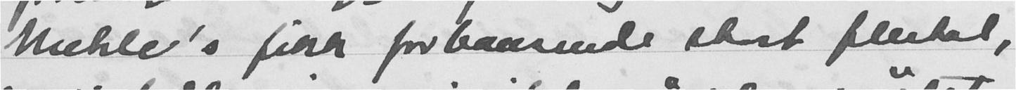Mehle's fikk forbausende stort flertal,
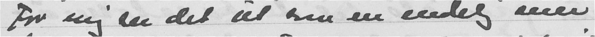For mig ser det ut som en endelig seier
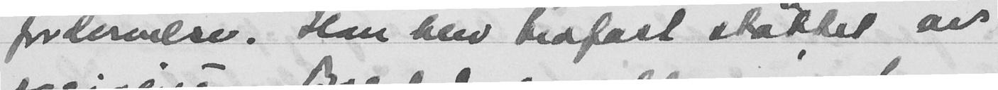fordervelse. Han blev trofast støttet av
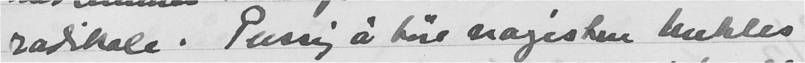radikale. Pussig å høre magister Mehles
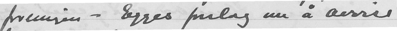foreningen - Egges forslag om å avvise
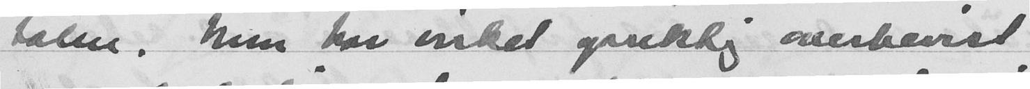talen. Men han virket opriktig overbevist
